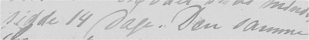sidde 14 Dage. Den samme
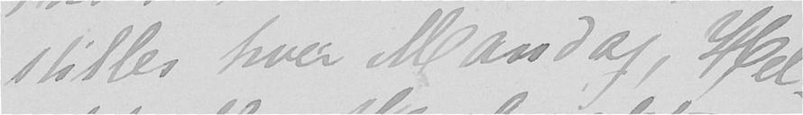stilles hver Mandag, Hel-
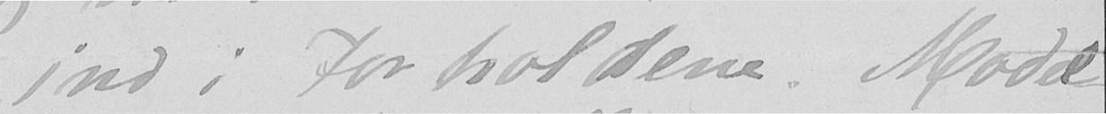ind i Forholdene. Model
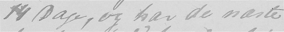14 Dage, og har de næste
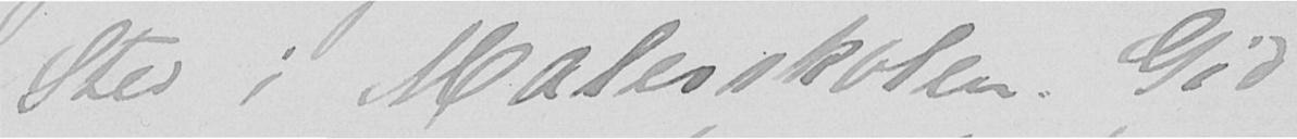Sted i Malerskolen. Gid
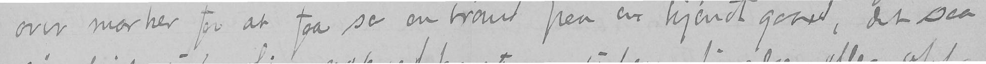over marker for at faa se en brand paa en kjendt gaard, det saa
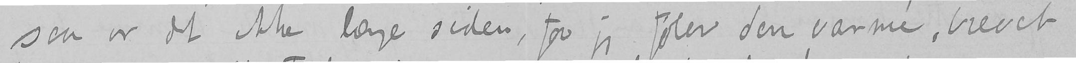saa er det ikke længe siden, for jeg føler den varme, brevet
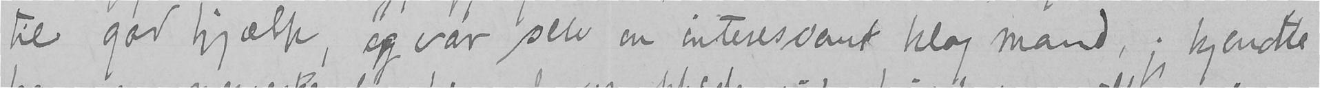til god hjælp og var selv en interessant klog mand, jeg kjendte
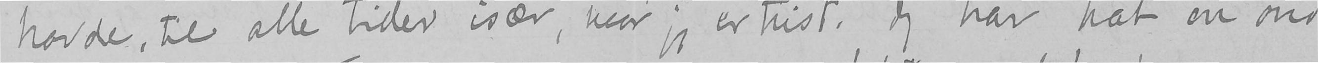havde, til alle tider især, naar jeg er trist. Jeg har hat en ond
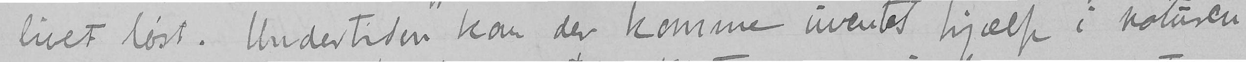livet løst. Undertiden kan der komme uventet hjælp i naturen
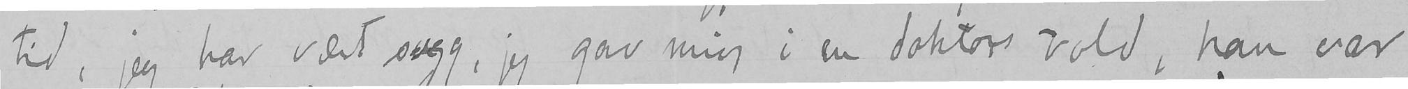tid, jeg har vært syg, jeg gav mig i en doktors vold, han var
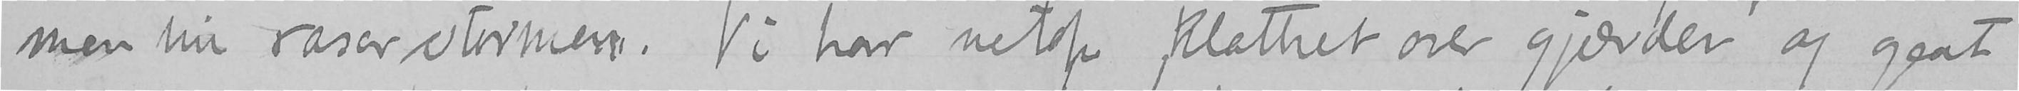men nu raser stormen. Vi har netop klattret over gjærder og gaat
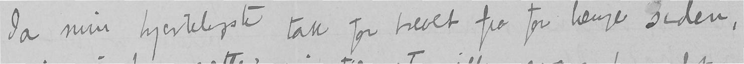Ja min hjerteligste tak for brevet fra for længe siden,
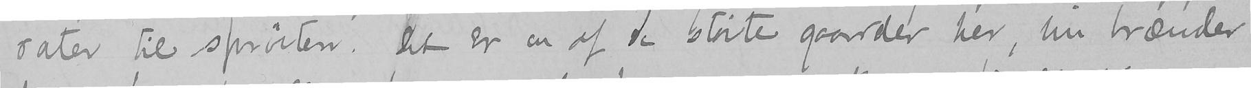rater til sprøiten. Det er en af de største gaarder her, nu brænder
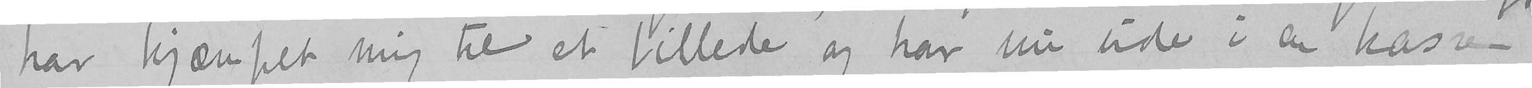har kjæmpet mig til et billede og har nu ude i en kasse
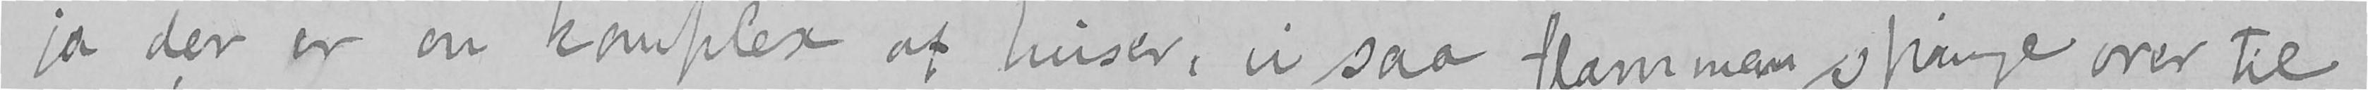ja der er en komplex af huser, vi saa flammen springe over til
